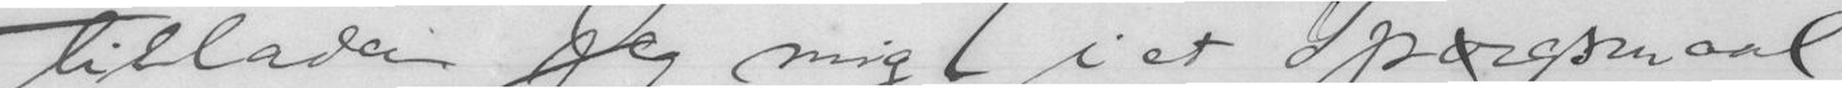Tillada jeg mig i et spørgsmaal
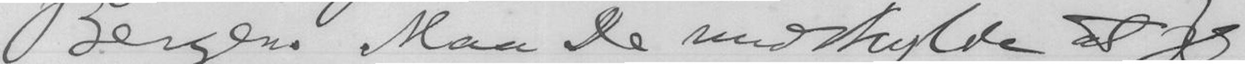Bergen. Maa de unnskylde at jeg
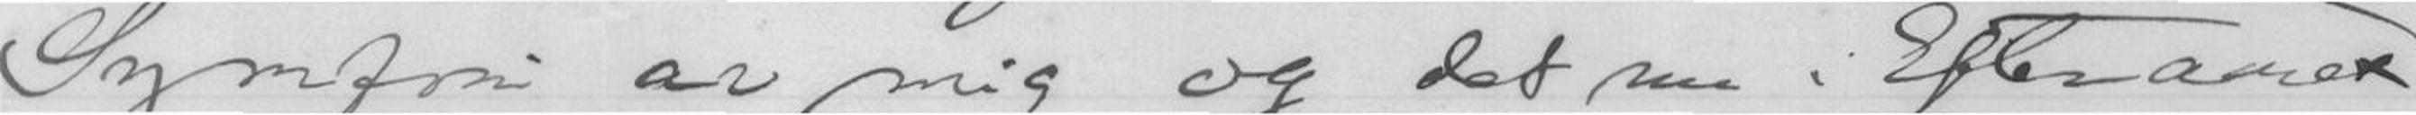Symfoni av mig og det nu i Efteraaret
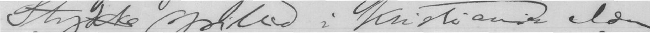Stykke spilled i Kristiania elder
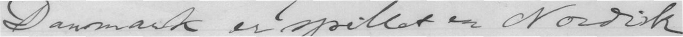Danmark er spillet en Nordisk
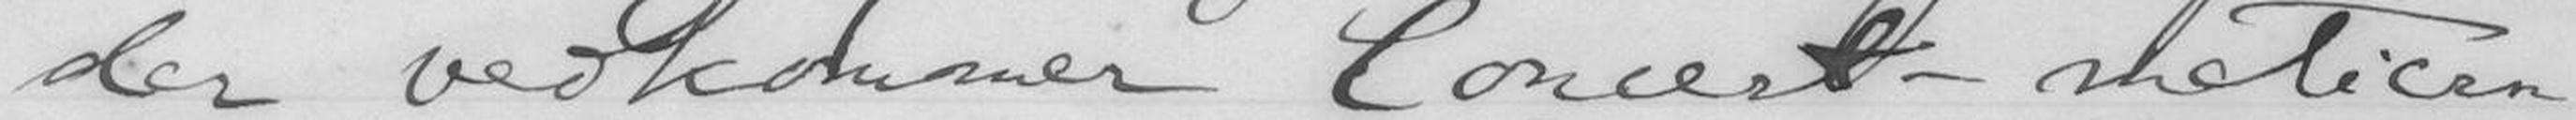der vedkommer Concert-metieren
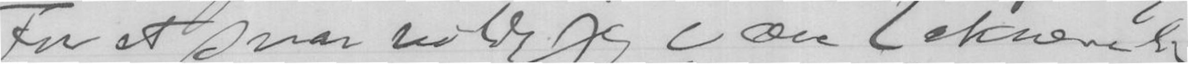For et svar vilde jeg vaere taknemlig
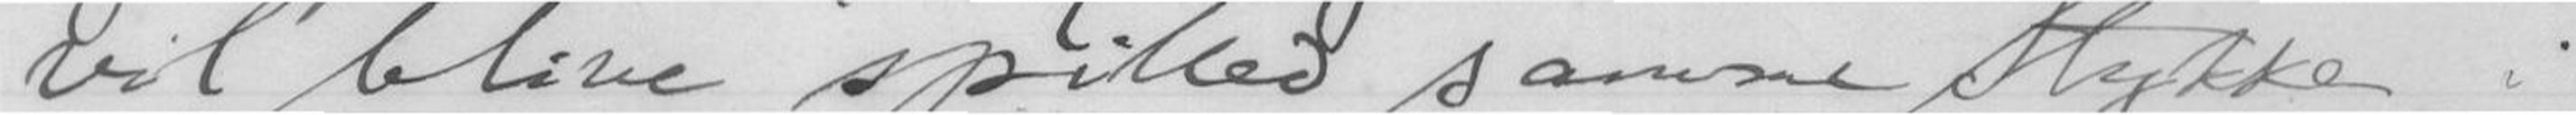vil blive spilled samme Stykke i
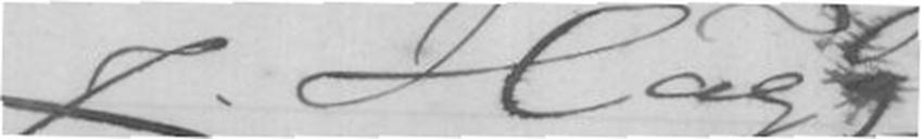J. Hägg
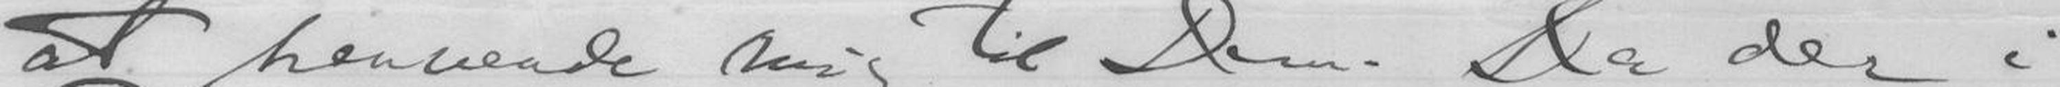At henvende mig til Dem. Da der i
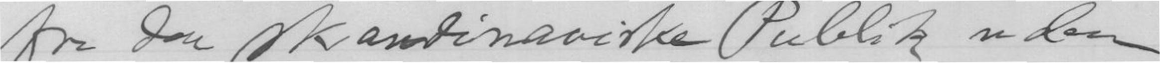for den skandinaviske Publik uden
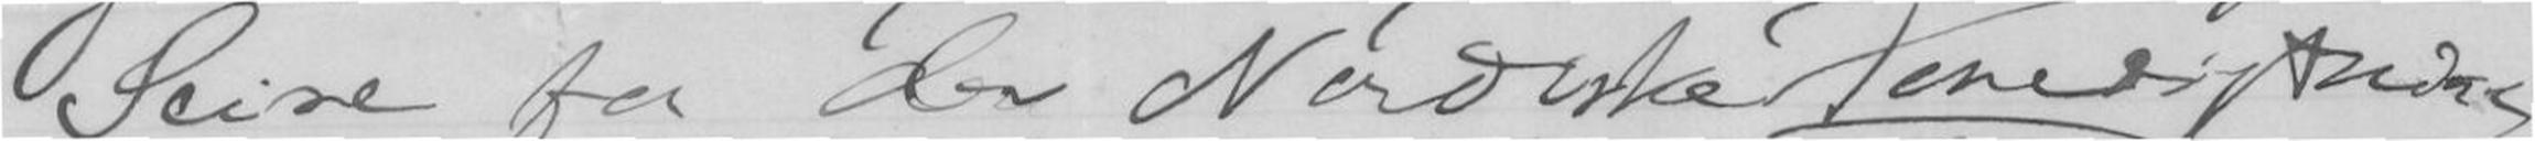Seire for den Nordiske Tonedigtning
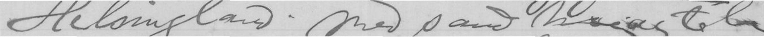Helsingland med sand høyagtelse
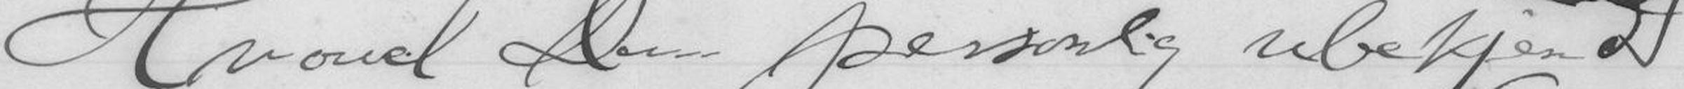Hvorved Dem Personlig ubekjendt
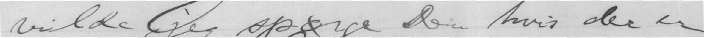vilde jeg spørge Dem hvis der er
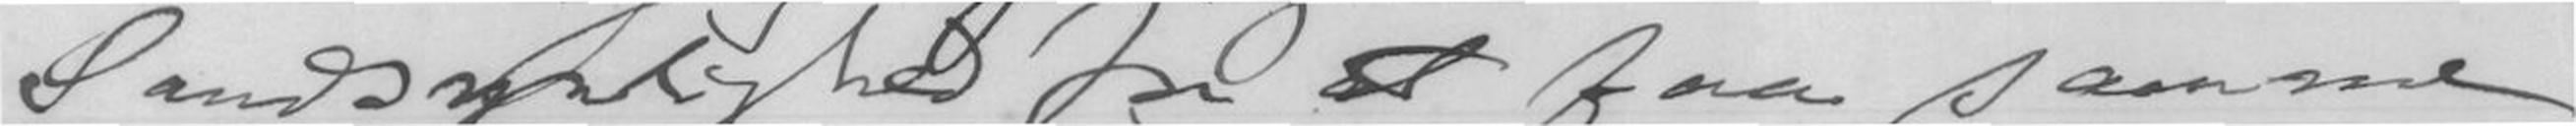Sandsynlighed att faa samme
